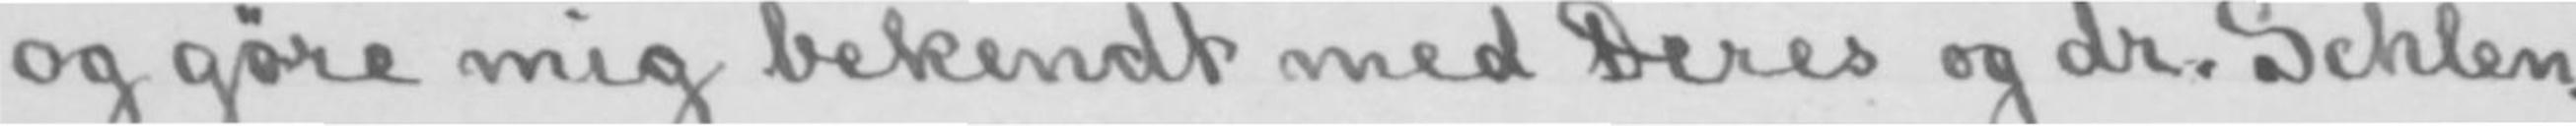og gøre mig bekendt med Deres og dr. Schlen-
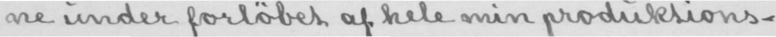ne under forløbet af hele min produktions-
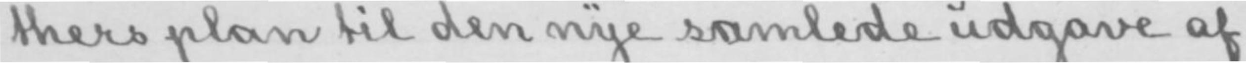thers plan til den nye samlede udgave af
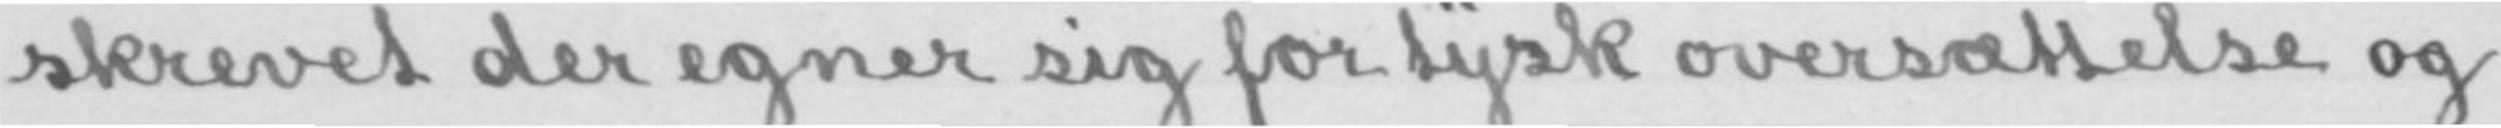skrevet der egner sig for tysk oversættelse og
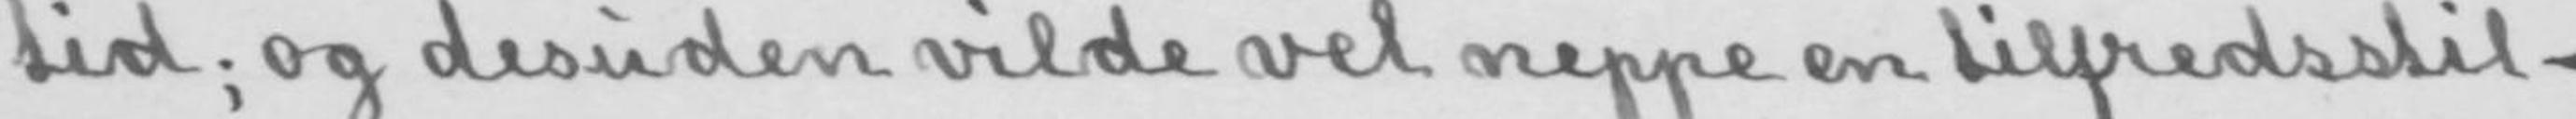tid; og desuden vilde vel neppe en tilfredsstil-
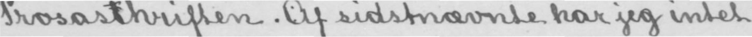Prosaschriften. Af sidstnævnte har jeg intet
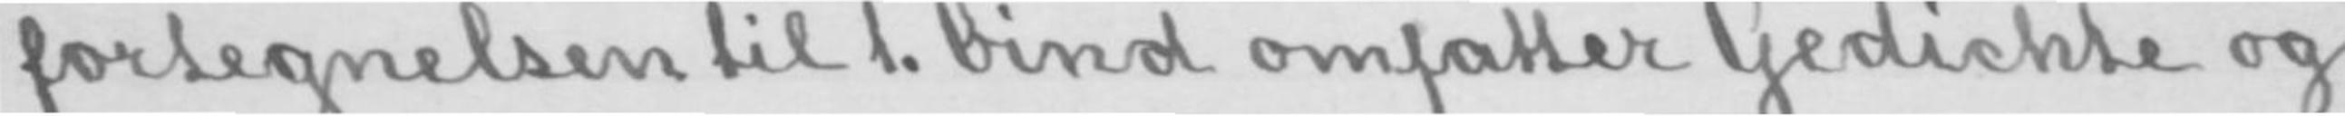fortegnelsen til 1. bind omfatter Gedichte og
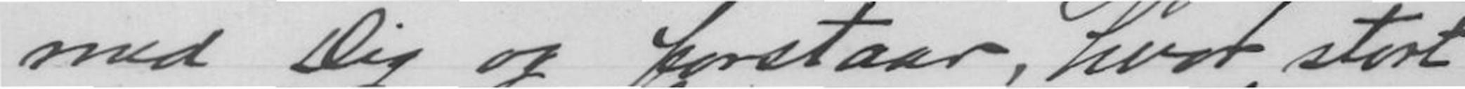med Dig og forstaar, hvor stort
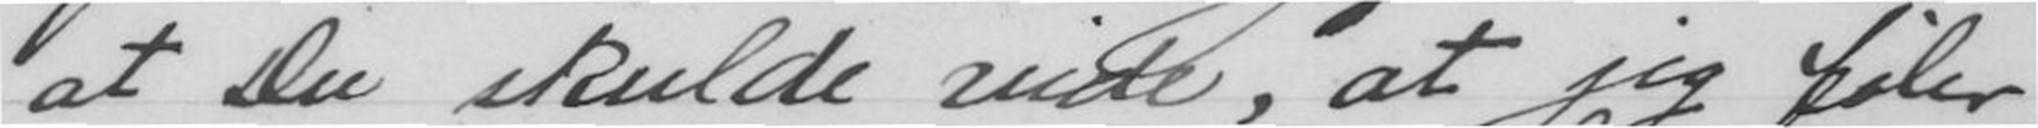at Du skulde vide, at jeg føler
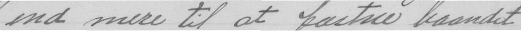end mere til at fæstne baandet
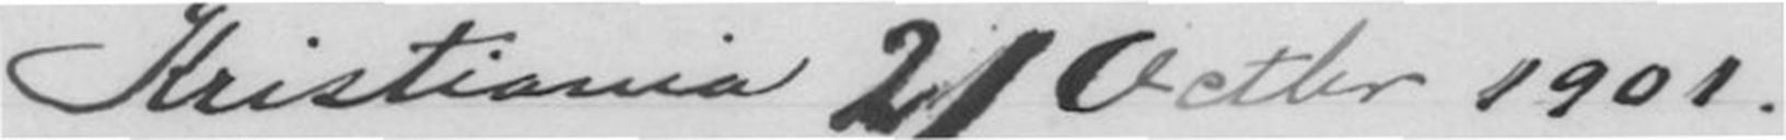Kristiania 21 Octbr 1901.
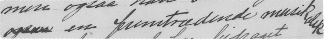ogsaa en fremtrædende musikalsk
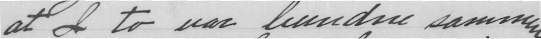at I to var bundne sammen,
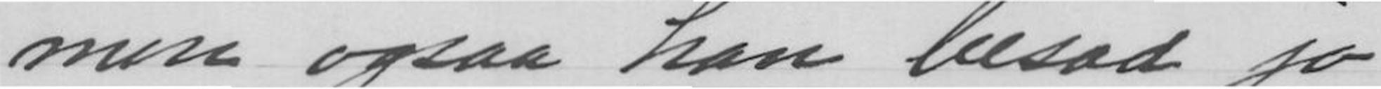men ogsaa han besad jo
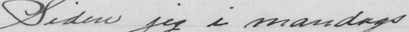Siden jeg i mandags
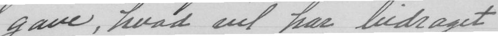gave, hvad vel har bidraget
